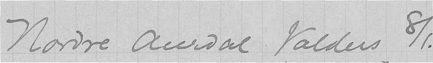Nordre Aurdal Valders 8/1.
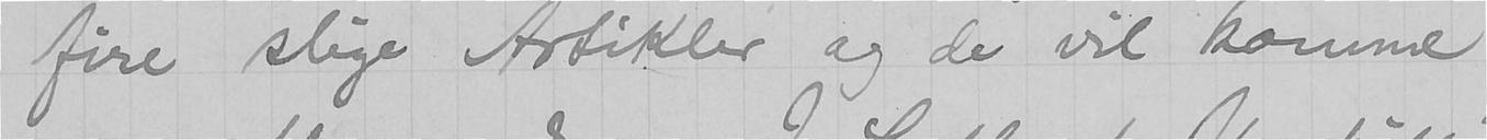fire slige Artikler og de vil komme
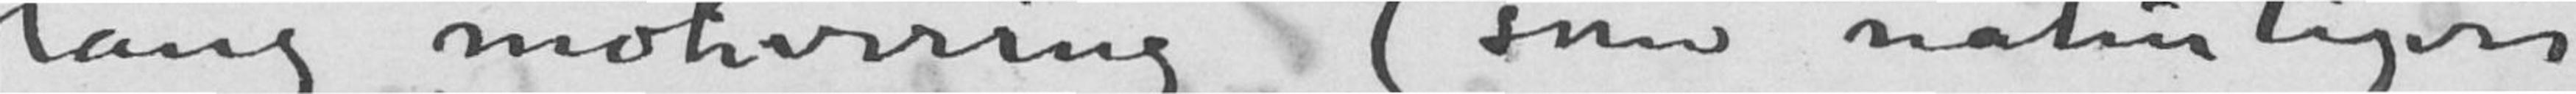lang motivering (som naturligvis
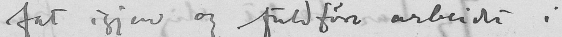fat igjen og fuldføre arbeidet i
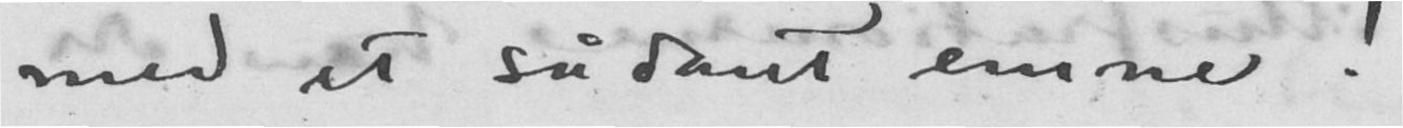med et sådant emne!
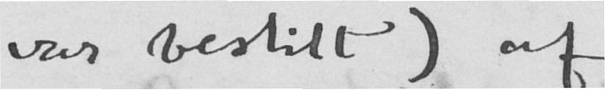var bestilt) af
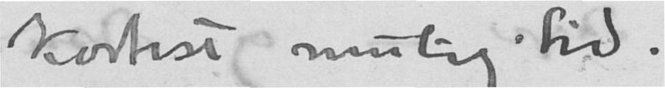kortest mulig tid.
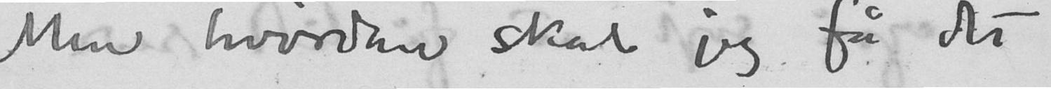Men hvordan skal jeg få det
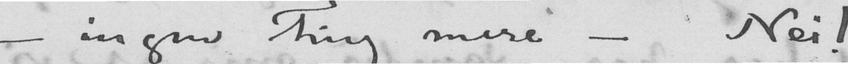- ingen ting mere - Nei!
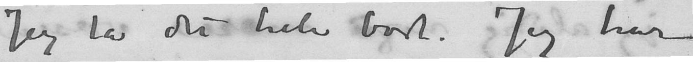Jeg la det hele bort. Jeg har
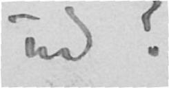ud?
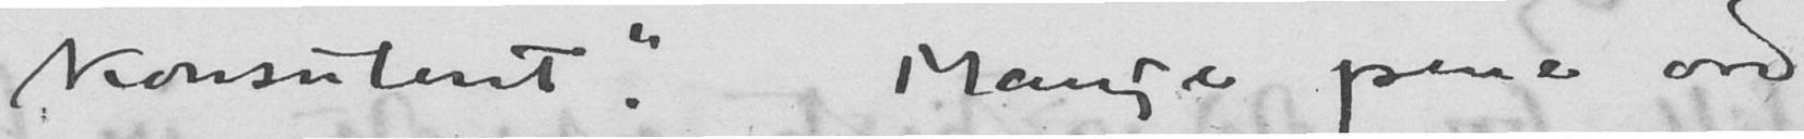konsulent". Mange pene ord
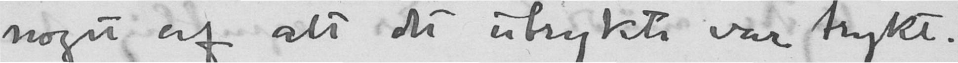noget af alt du utrykte var trykt.
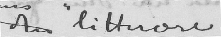de "litterære
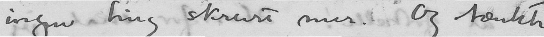ingen ting skrevet mer. Og tænkte
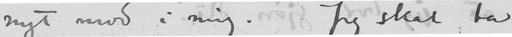nyt mod i mig. Jeg skal ta
